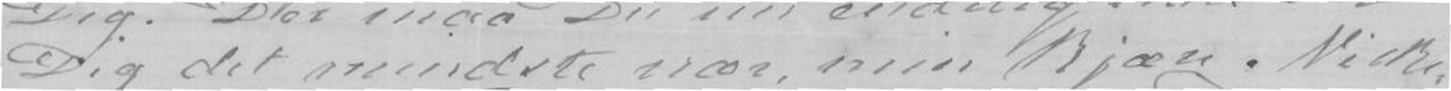Dig det mindste nær, min kjære Niske,
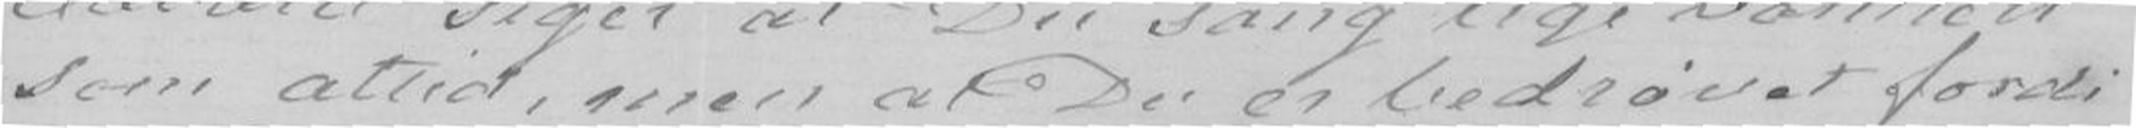som altid, men at Du er bedrøvet fordi
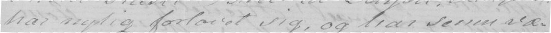har nylig forlovet sig, og har senere væ-
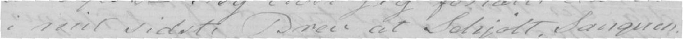i mit sidste Brev at Schjøtt Sangeren
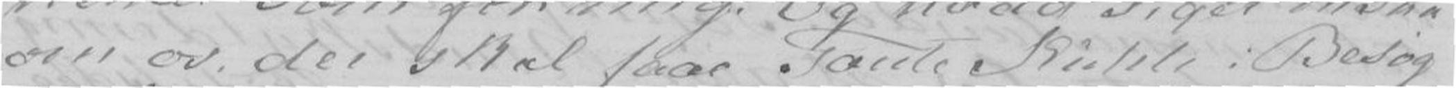om os der skal faae tante Kühle i Besøg
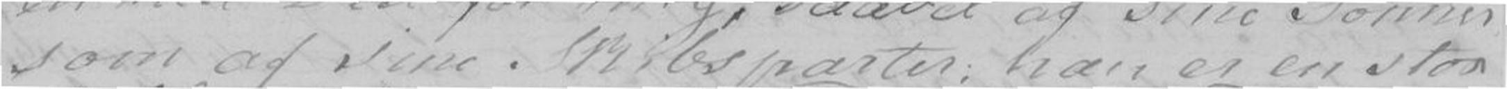som af sine Skibsparter, han er en stor
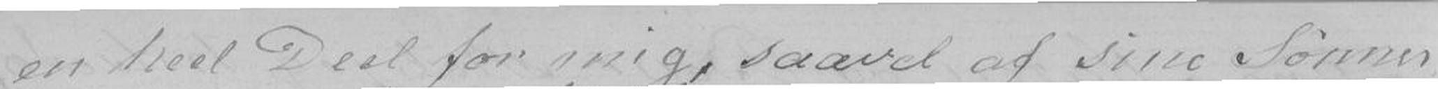en haal Deel for mig, saavel af sine Sønner
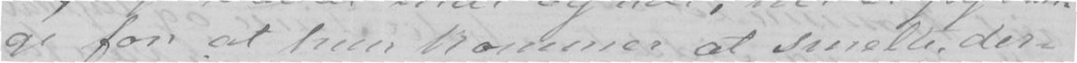ge for at hun kommer at smelte der-
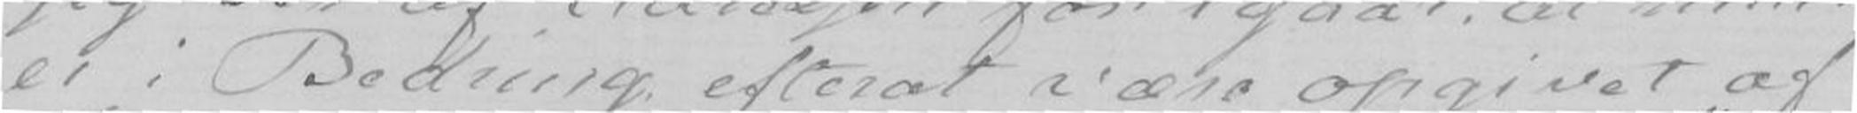er i Bedring efterat være opgivet af
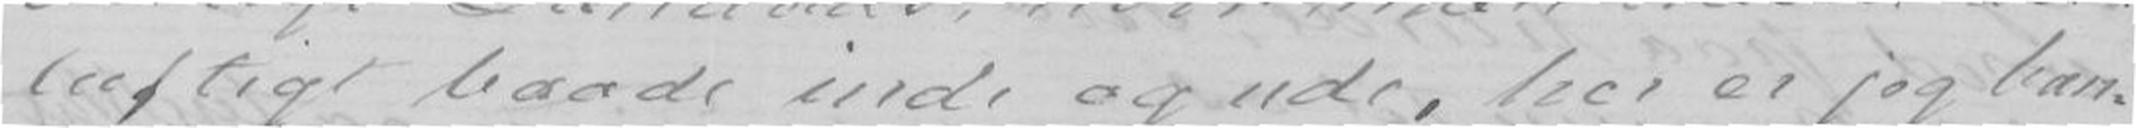Luftig baade inde og ude, her er jeg ban-
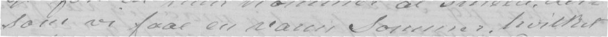som vi faar en varm Sommer, hvilket
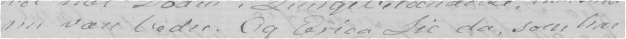nu være bedre. Og Erica Lie da, som har
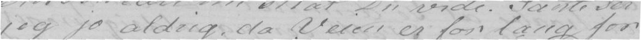jeg jo aldrig, da Veien er for lang for
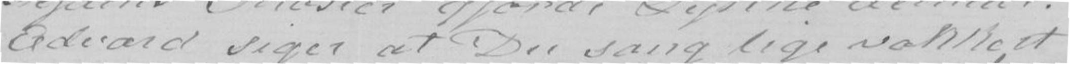Edvard siger at Du sang lige vakkert
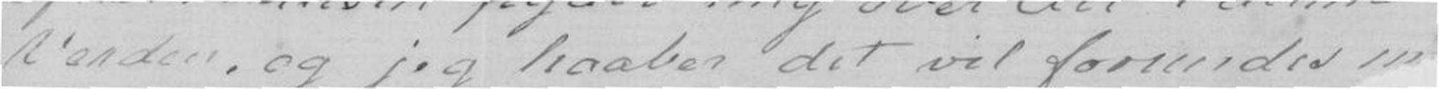Verden, og jeg haaber det vil forundes m
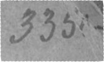335.
